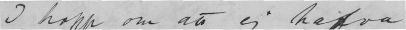I hopp om att ej hafva
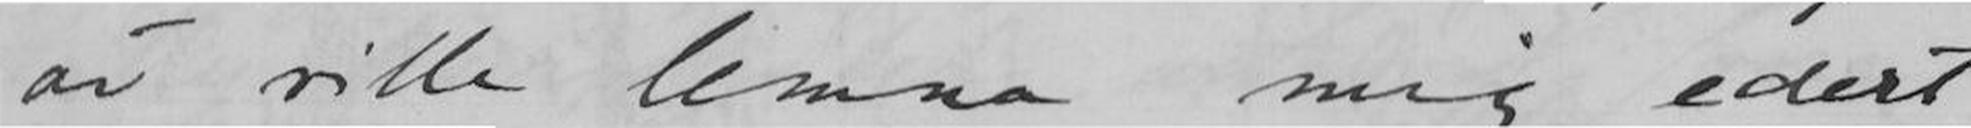att ville lemna mig edert
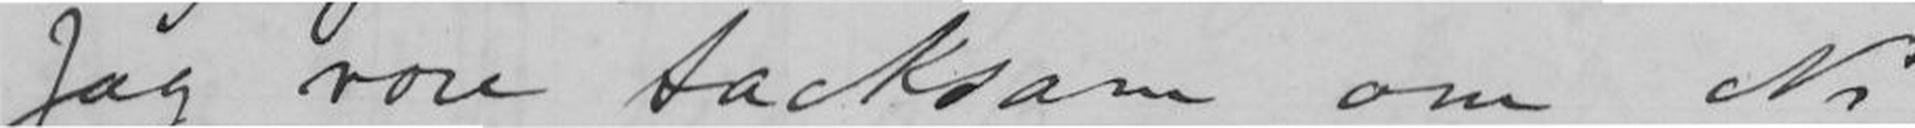Jag vore tacksam om Ni
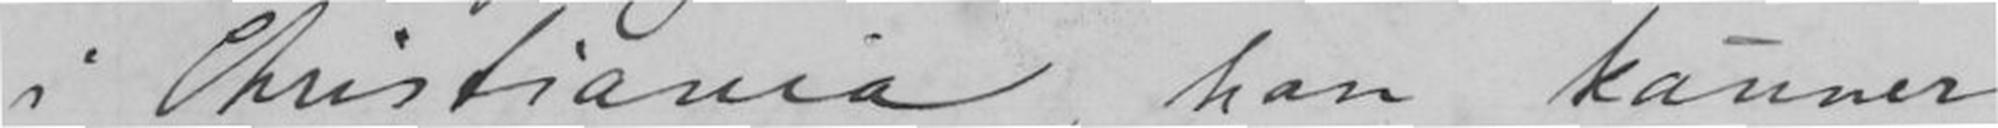i Christiania, han känner
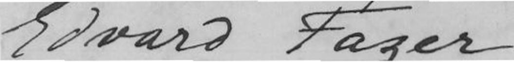Edvard Fazer
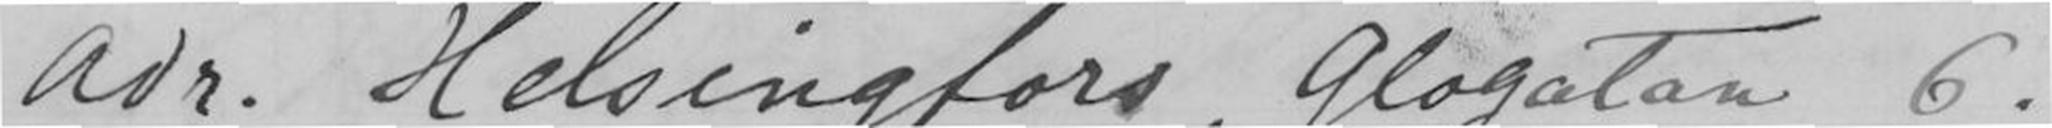Adr. Helsingfors, Glogatan 6.
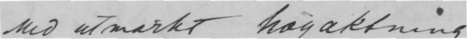Med utmärkt högaktning
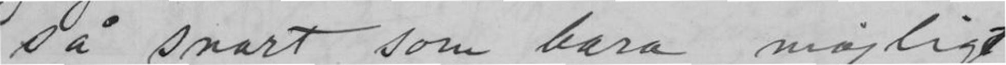så snart som bara möjligt
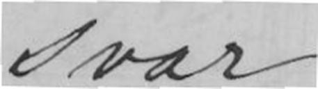svar.
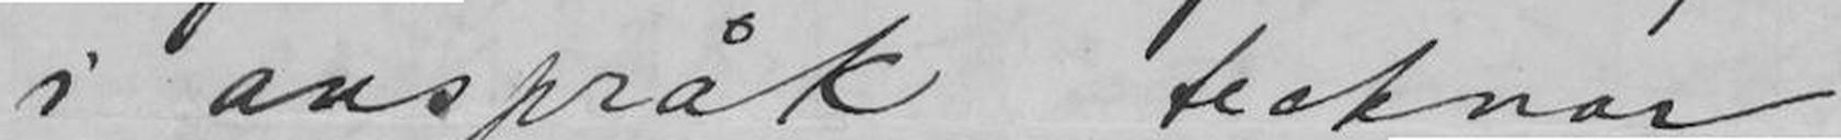i anspråk tecknar
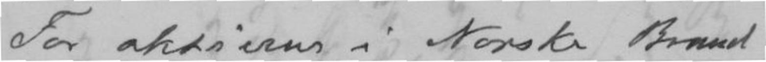For aktivens i Norske Brand
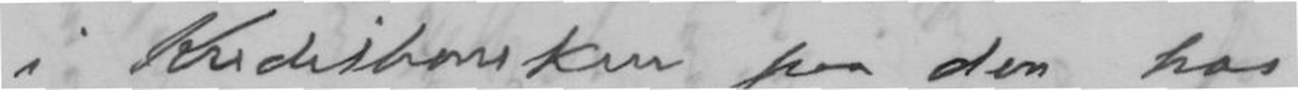i Kreditbanken paa den hos
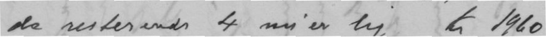de resterende 4 nu er lig kr 1960
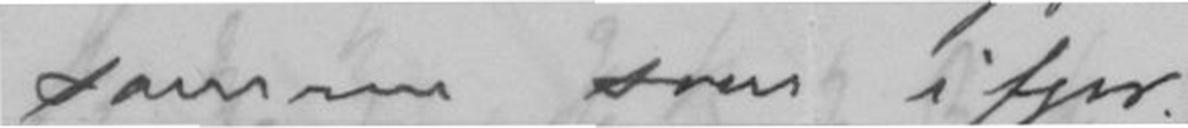samme som ifjor.
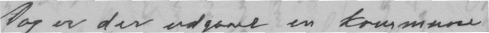Dog er der udgaaet en kommune-
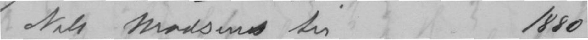Nils Madsens til1880
